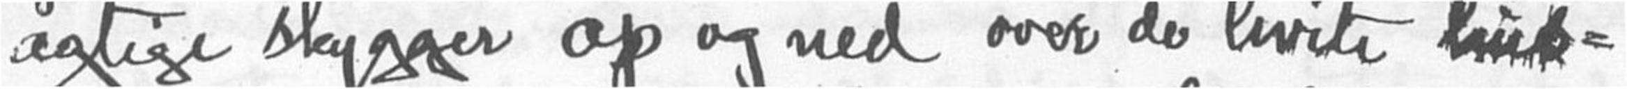agtige skygger op og ned over de hvite hus-
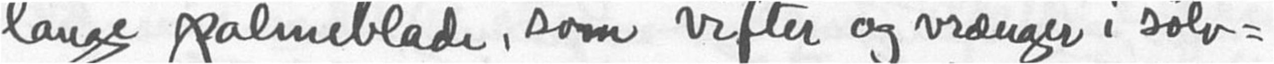lange palmeblade, som vifter og vrænger i sølv-
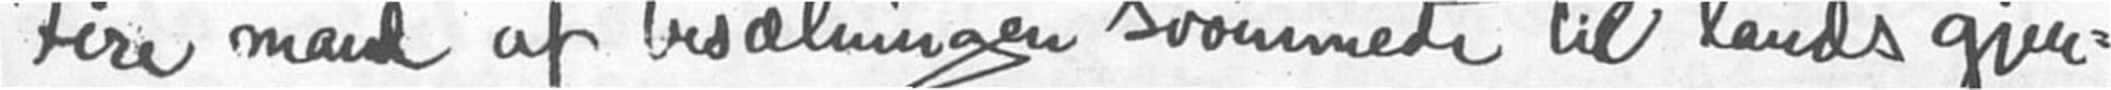Fire mand af besætningen svømmede til lands gjen-
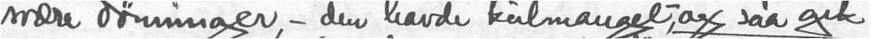svære dønninger, - den havde kulmangel, og saa gik
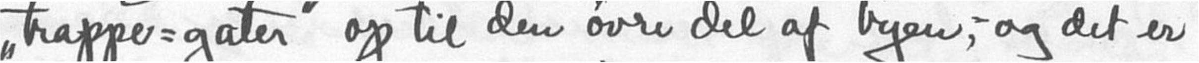"trappe-gater" op til den øvre del af byen, - og det er
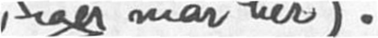siger man her).
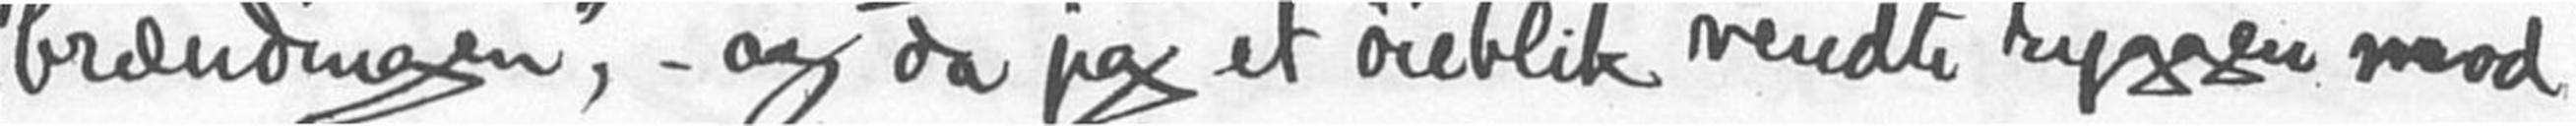"brændingen", - og da jeg et øieblik vendte ryggen mod
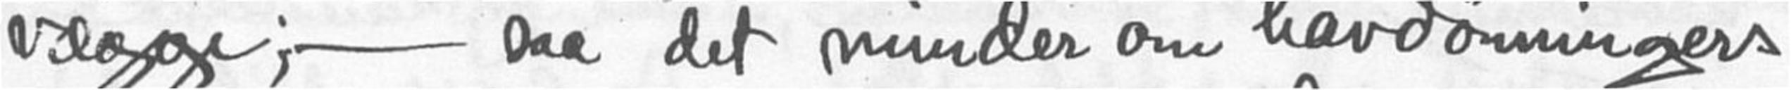vægge; - saa det minder om havdonningernes
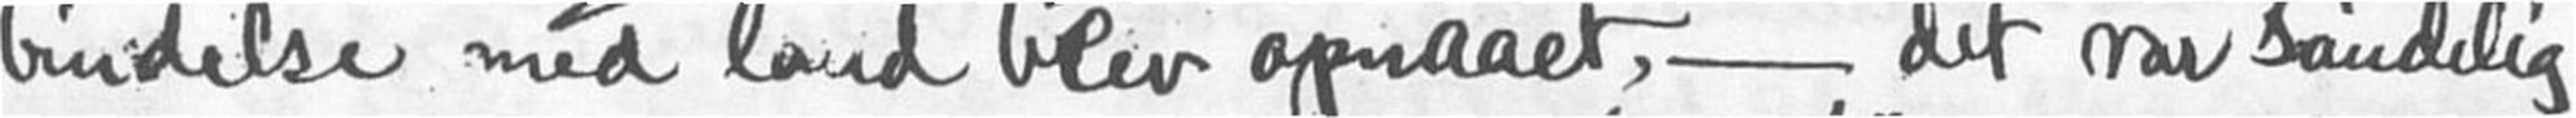bindelse med land blev opnaaet, - det var sandelig
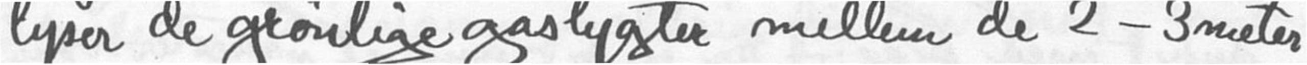lyser de grønlige gaslygter mellem de 2-3 meter
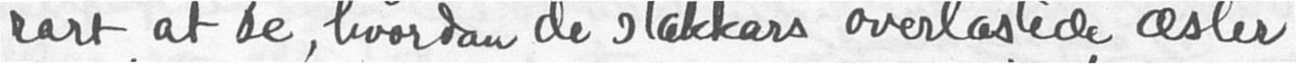rart at se, hvordan de stakkars overlastede æsler
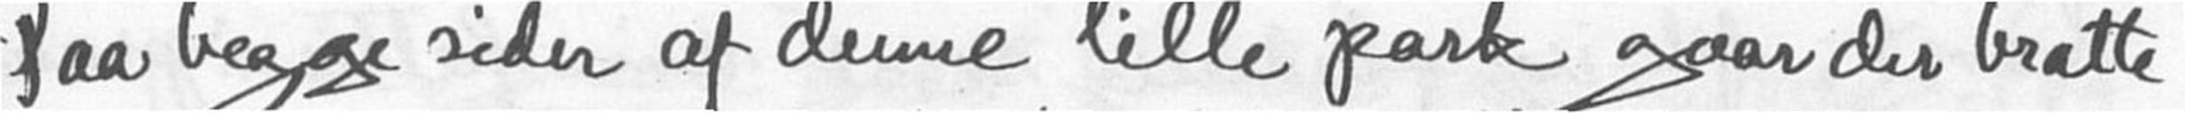Paa begge sider af denne lille park gaar der bratte
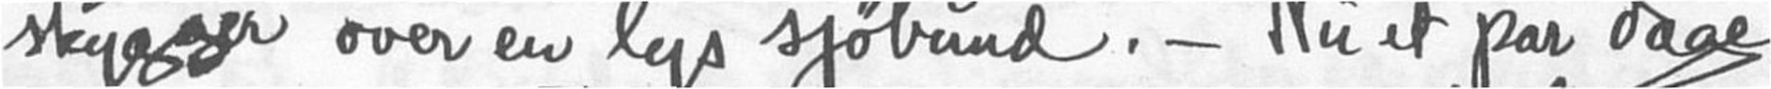skygge over en lys sjøbund. - Nu et par dage
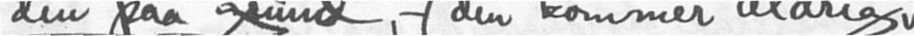den paa grund, - (den kommer aldrig
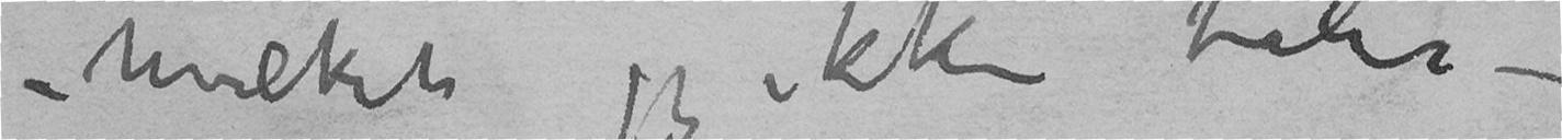– hvilket jeg ikke tåler –
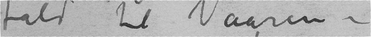fald til Vaaren –
